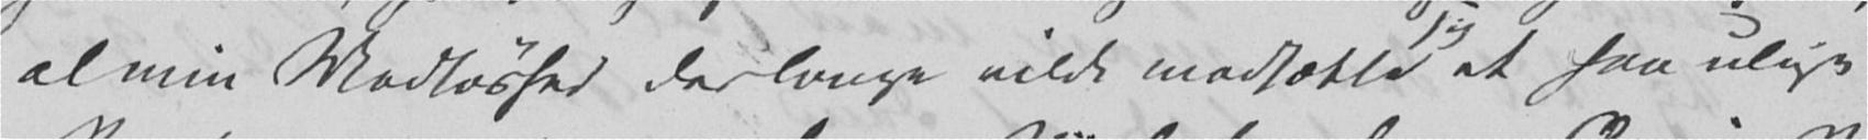al min Modløshed der længe vilde modsætte sig et saa ulige
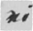vi
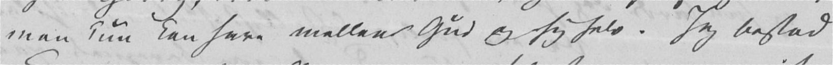man kun kan have mellem Gud og sig selv. Jeg bestod
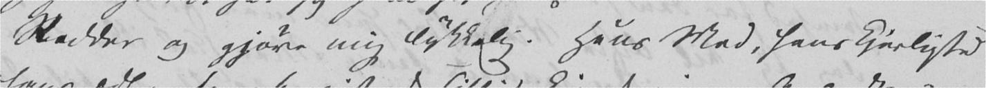Redder og gjøre mig lykkelig. Hans Mod, hans Kjerlighed
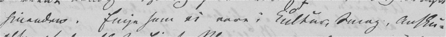hinanden. Enige som vi vare i Kultur, Smag, Ansku-
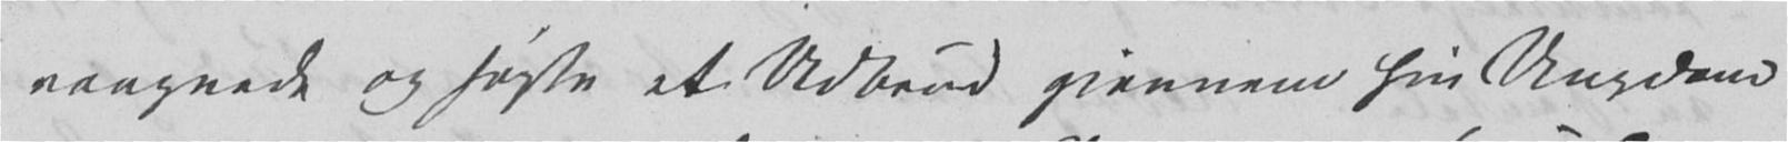vaagnede og søgte et Udbrud gjennem sin Ungdom
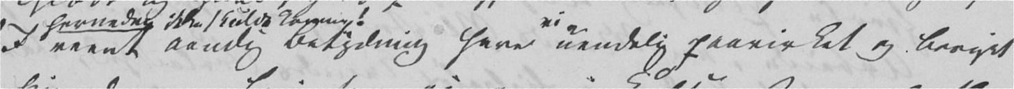I reent aandig Betydning have vi uendelig paavirket og beriget
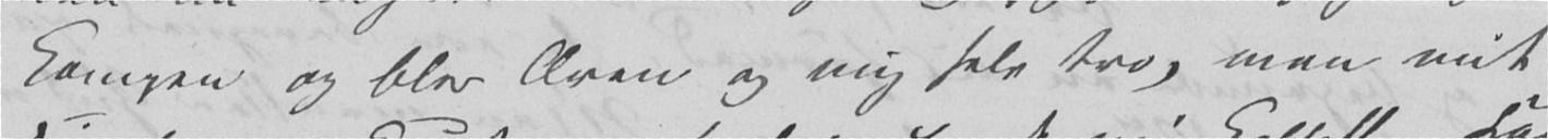Kampen og blev Æren og mig selv tro, men mit
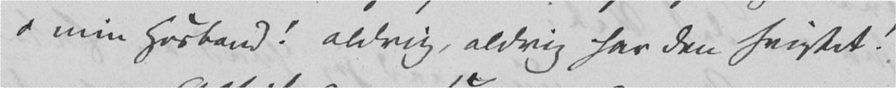o min Husbond! aldrig, aldrig har den svigtet!
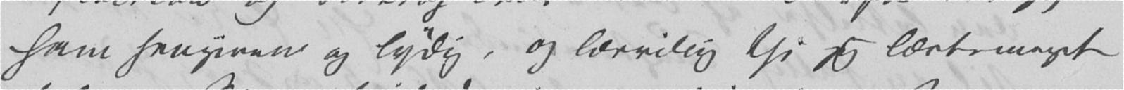ham hengiven og lydig, og lærvillig thi jeg lærte meget
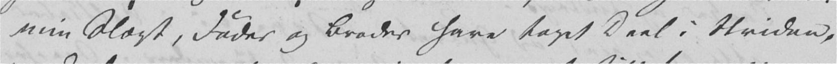min Slægt, Fader og Broder have taget Deel i Striden,
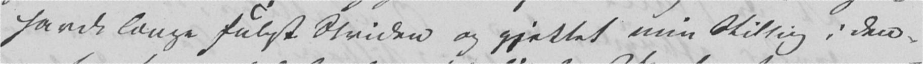havde længe fulgt Striden og gjettet min Stilling i den
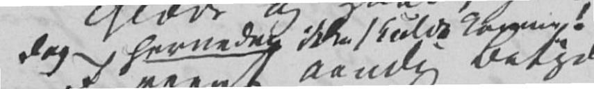dog hernede ikke skulde komme!
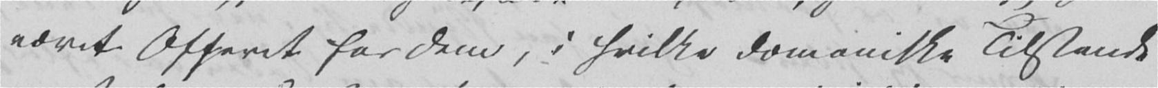været Offeret for dem, i hvilke dæmoniske Tilstande
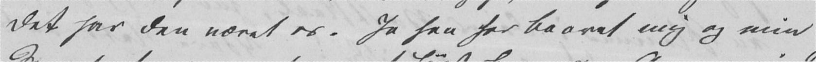det har den været os. Ja han har baaret mig og min
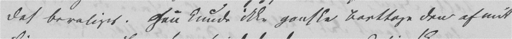det beroliges. Han kunde ikke ganske borttage den af mit
